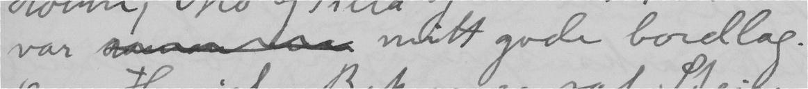var  mitt gode bordlag.
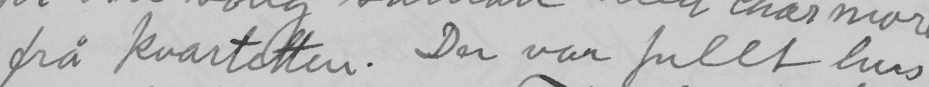frå kvartetten. Der var fullt hus
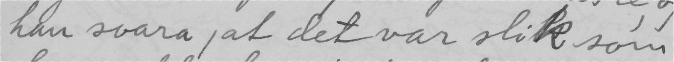han svara, at det var slik som
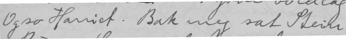Ogso Harriet. Bak meg sat Stein
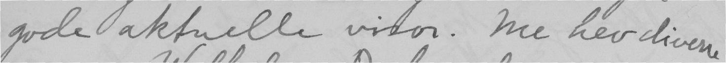gode aktuelle visor. Me hev diverre
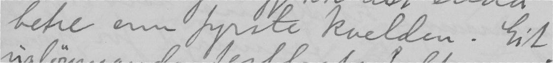betre enn fyrste kvelden. Eit
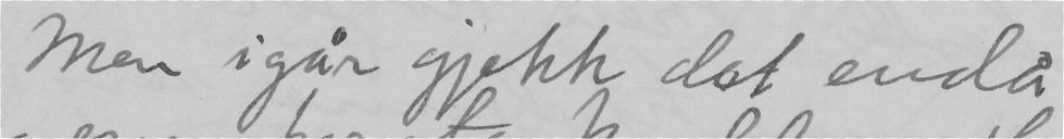Men igår gjek det endå
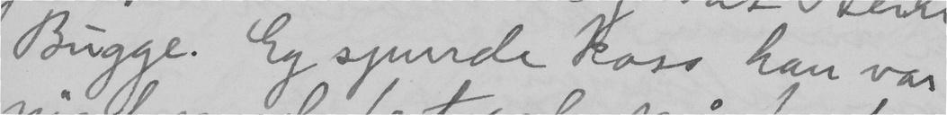Bugge. Eg spurde koss han var
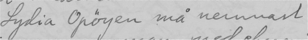Lydia Opöyen må nemnast
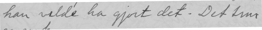han vilde ha gjort det. Det trur
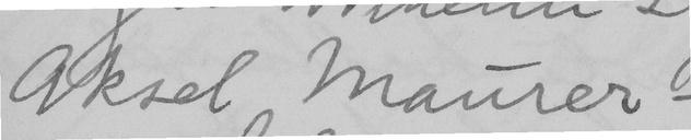Aksel Maurer –
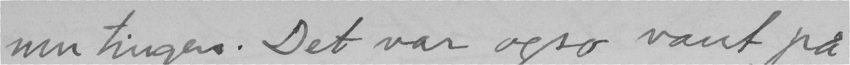um tingen. Det var ogso vant på
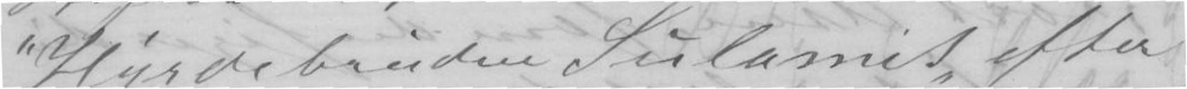Hyrdebruden Sulamit efter
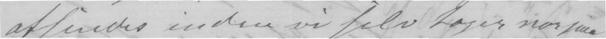afindes inden vi selv tager norsine
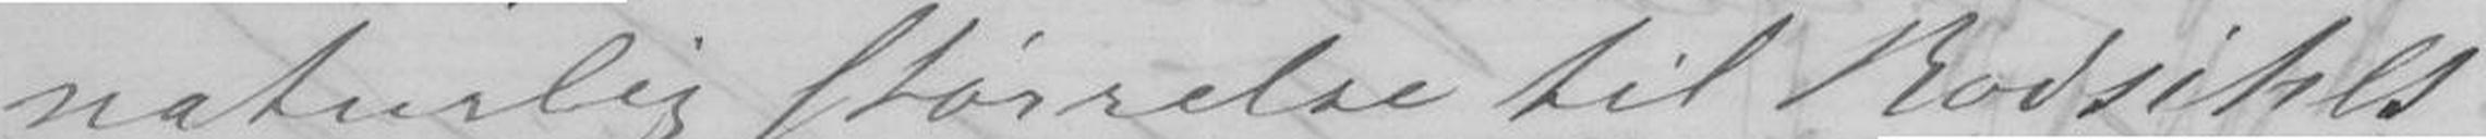naturlig størrelse til Bodsihls
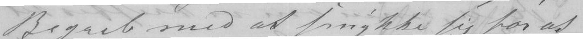Begreb med at smykke sig for at
